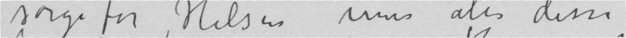sørge for Helsen min alle disse
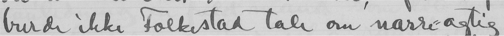burde ikke Folkestad tale om narre-agtig
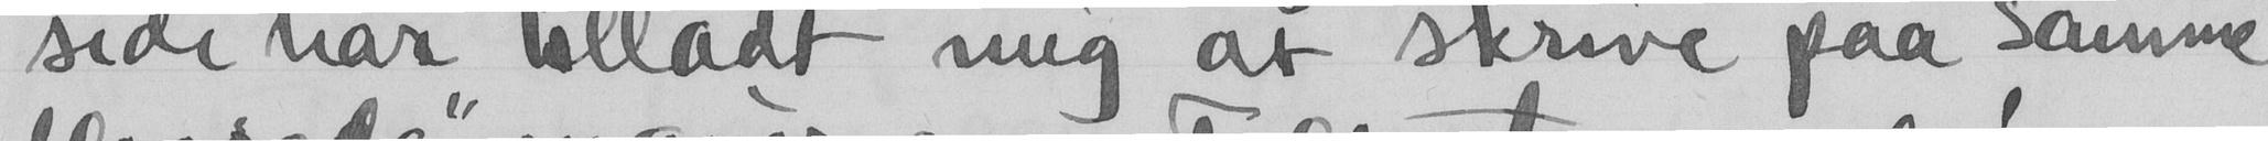side har tilladt mig at skrive paa samme
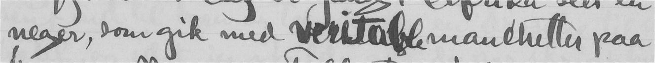neger, som gik med veritable manchetter paa
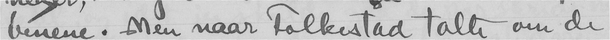benene. Men naar Folkestad talte om de
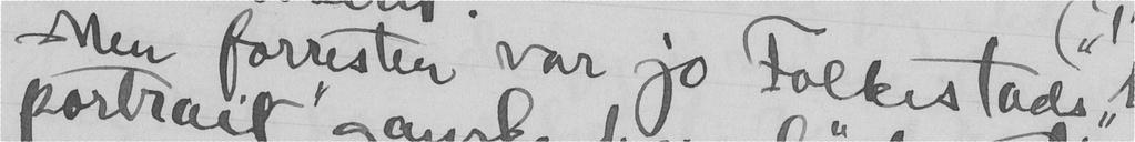Men forresten var jo Folkestads
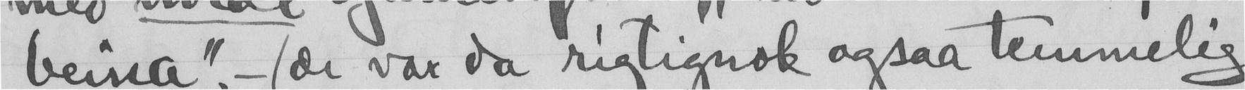beina" - (de var da rigtignok ogsaa temmelig
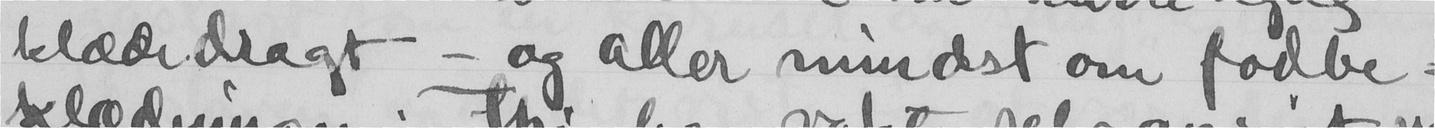klædedagt - og aller mindst om fodbe-
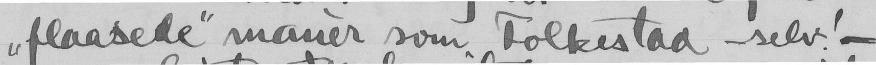"flaasede" maner som Folkestad selv! -
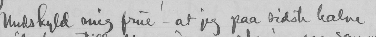undskyld mig frue - at jeg paa sidste halve
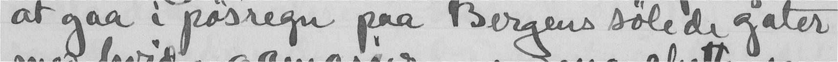at gaa i pøsregn paa Bergens sølede gater
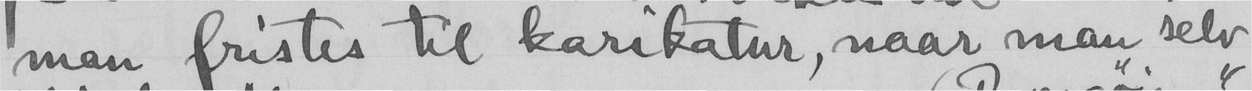man fristes til karikatur, naar man selv
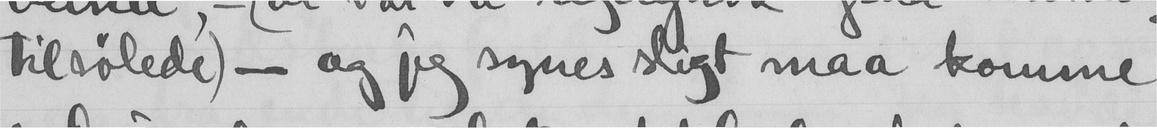tilsølede) - og jeg synes sligt maa komme
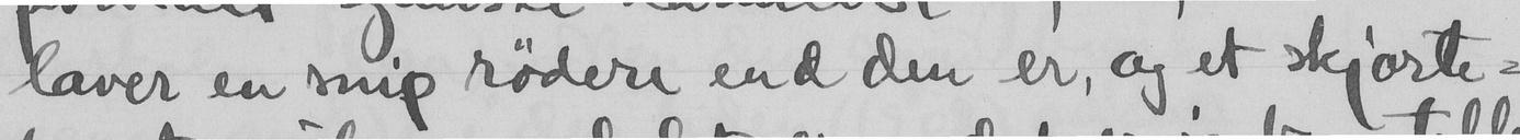laver en snip rødere end den er, og et skjorte-
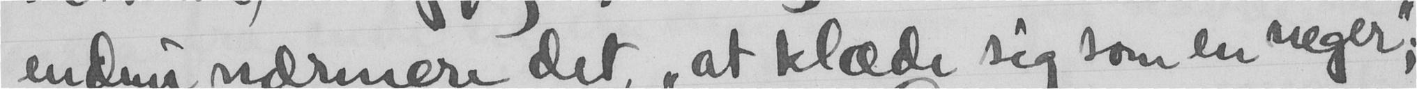endnu nærmere det, "at klæde sig som en neger";
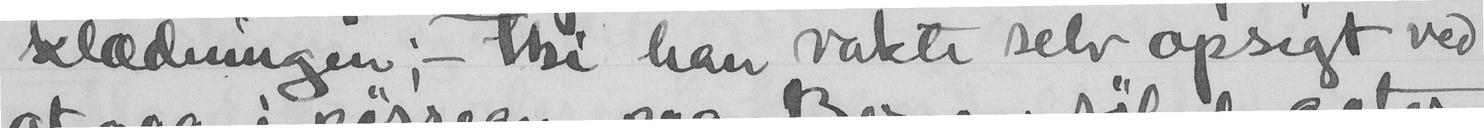klædningen; - thi han vakte selv opsigt ved
